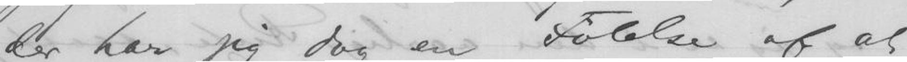der har jeg dog en Følelse af at
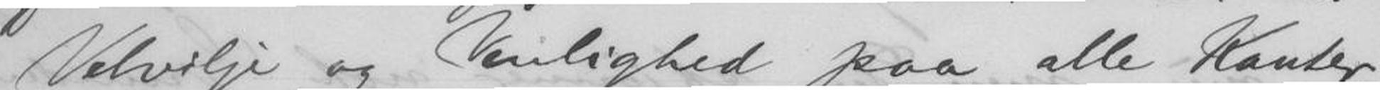Velvilje og Venlighed paa alle Kanter,
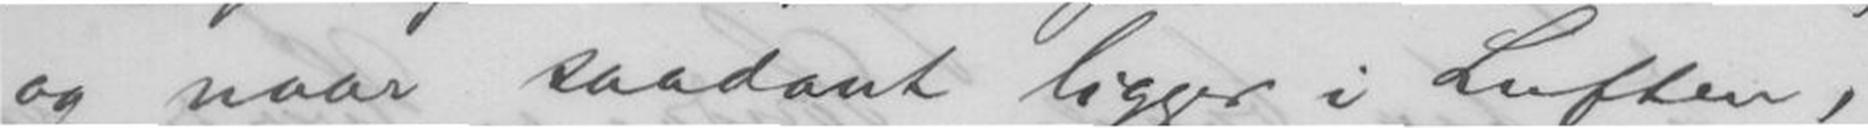og naar saadant ligger i Luften,
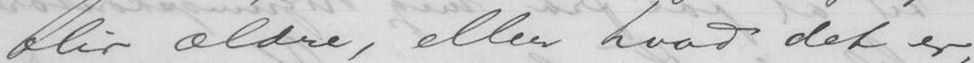blir ældre, eller hvad det er,
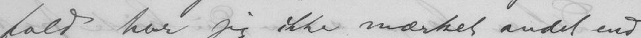fald har jeg ikke mærket andet end
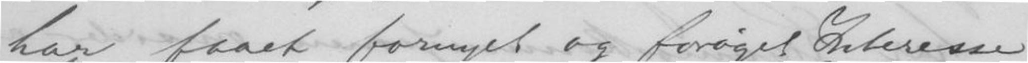har faaet fornyet og forøget Interesse
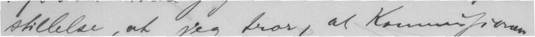stillelse, at jeg tror, at Kommissionen
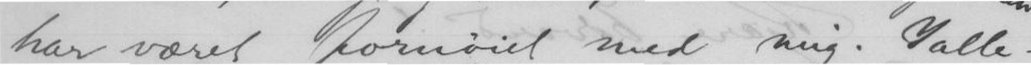har været fornøiet med mig. Ialle-
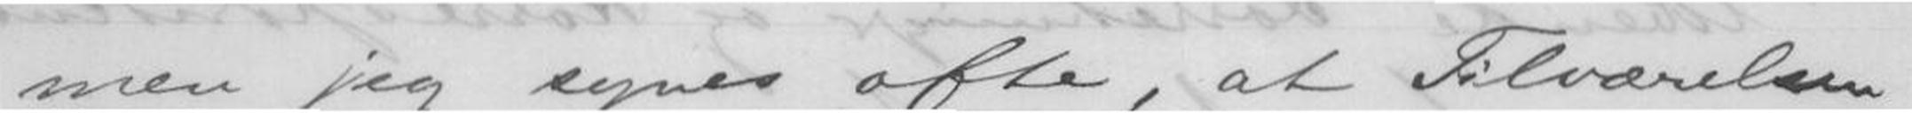men jeg synes ofte, at Tilværelsen
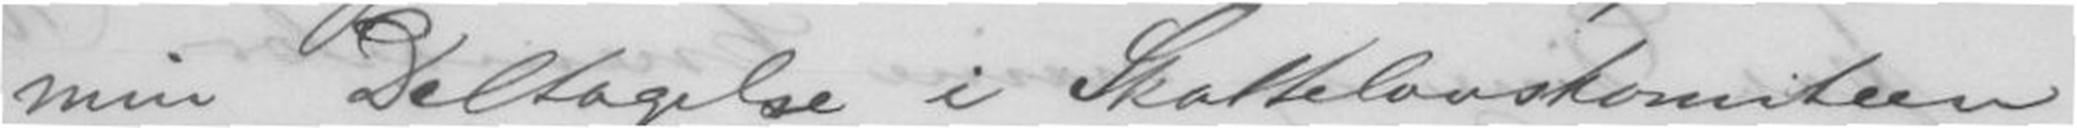min Deltagelse i Skattelovskomiteen
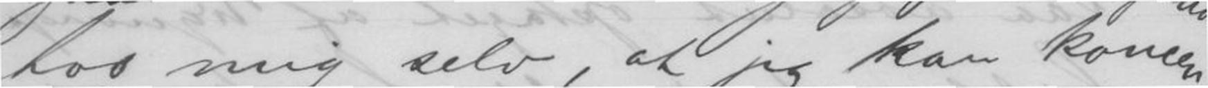hos mig selv, at jeg kan koncen-
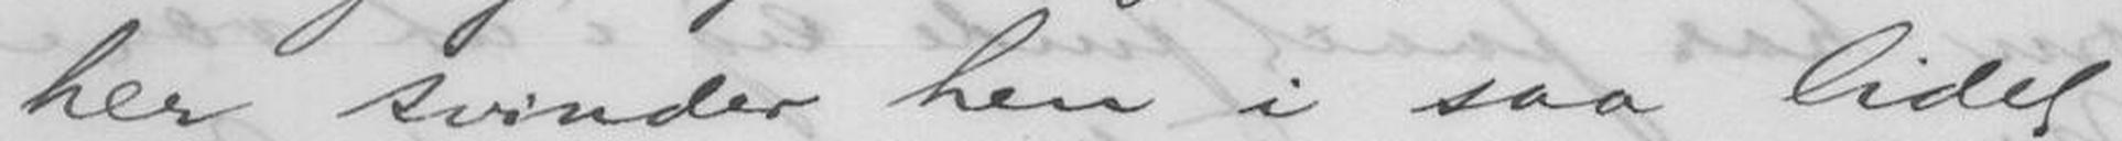her svinder hen i saa lidet,
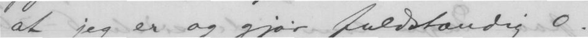at jeg er og gjør fuldstændig 0.
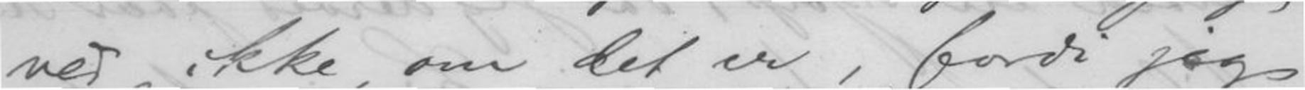ved ikke, om det er, fordi jeg
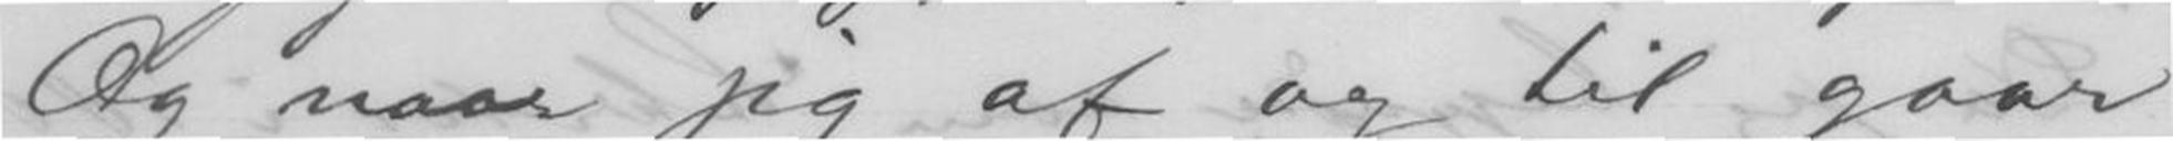Og naar jeg af og til gaar
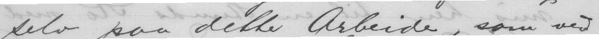selv paa dette Arbeide, som ved
